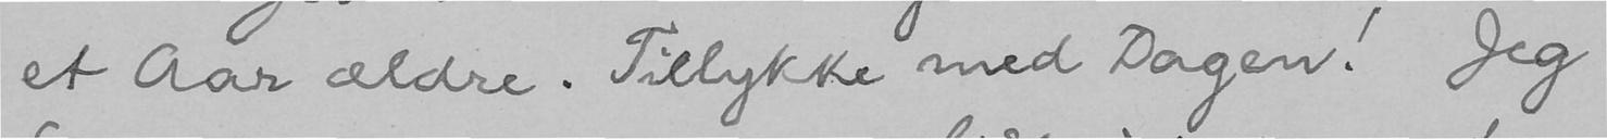et Aar ældre. Tillykke med Dagen! Jeg
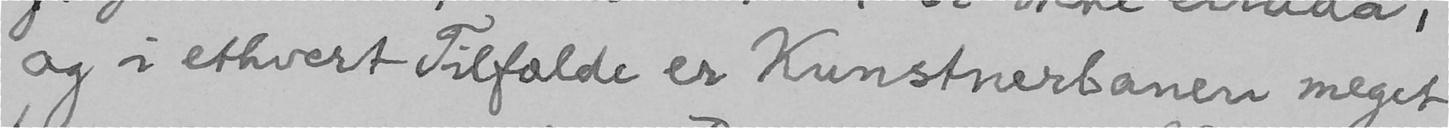og i ethvert Tilfælde er Kunstnerbanen meget
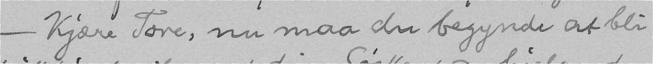- Kjære Tore, nu maa du begynde at bli
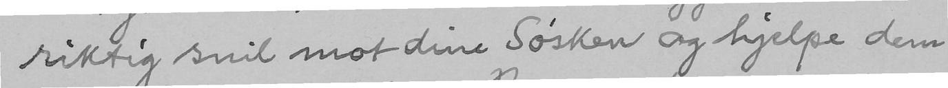riktig snil mot dine Søsken og hjelpe dem
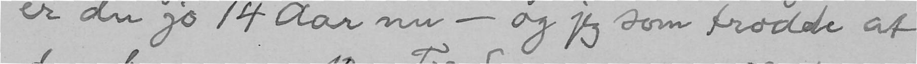er du jo 14 Aar nu - og jeg som trodde at
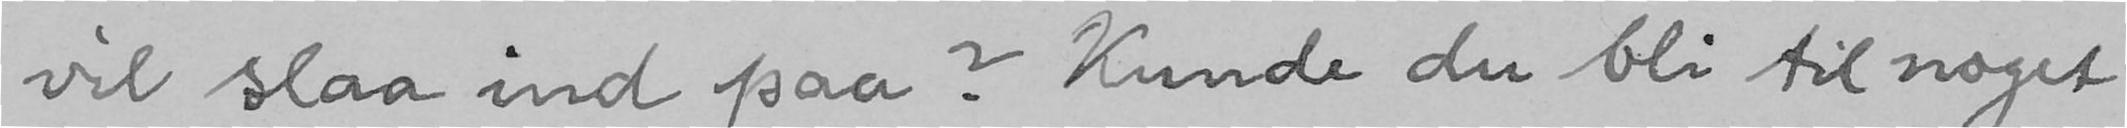vil slaa ind paa? Kunde du bli til noget
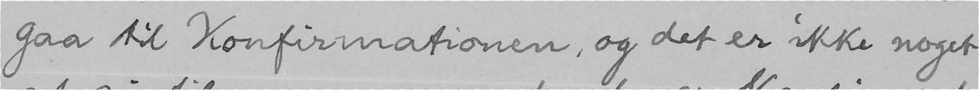gaa til Konfirmationen, og det er ikke noget
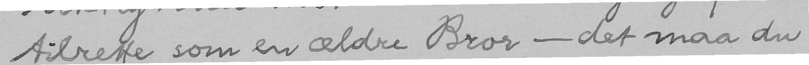tilrette som en ældre Bror - det maa du
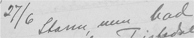27/6 Storm, men bad
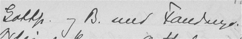Gottfr. og B. med Fandango.
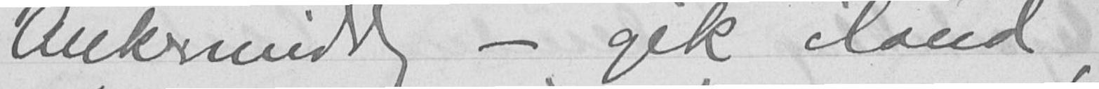Ankermiddag - gik iland,
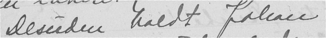Desuden holdt Johan
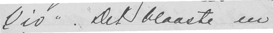"Viv". Det blaaste en
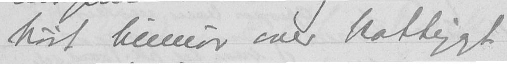høit humør over nattejagt
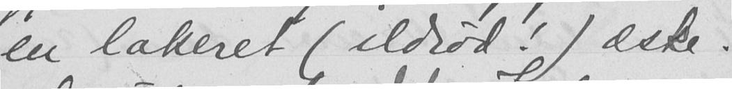en lakeret (ildrød!) æske.
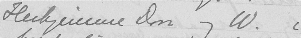Herhjemme Don og W. i
